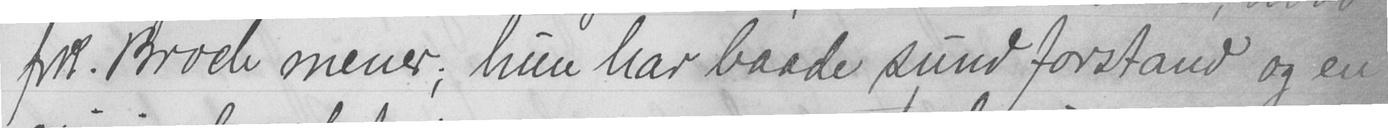frk. Broch mener; hun har baade sund forstand og en
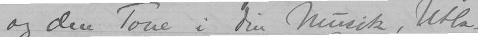og den Tone i Din Musik, Utla-
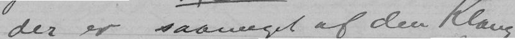der er saameget af den Klang
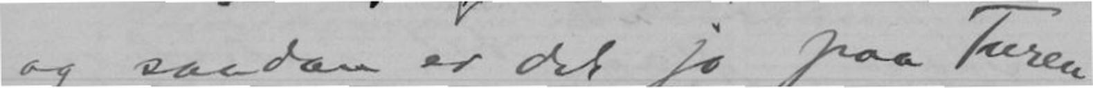og saadan er det jo paa Turen
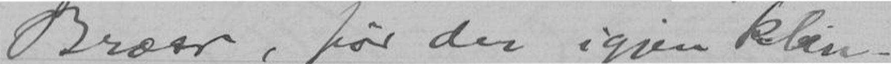Bræer, før der igjen klin-
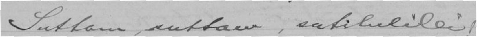Suttam, suttam, sutilulilei!
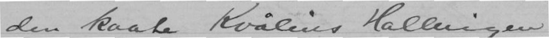den kaate Kvålins Hallingen
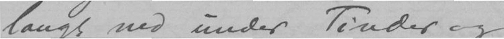langt ned under Tinder og
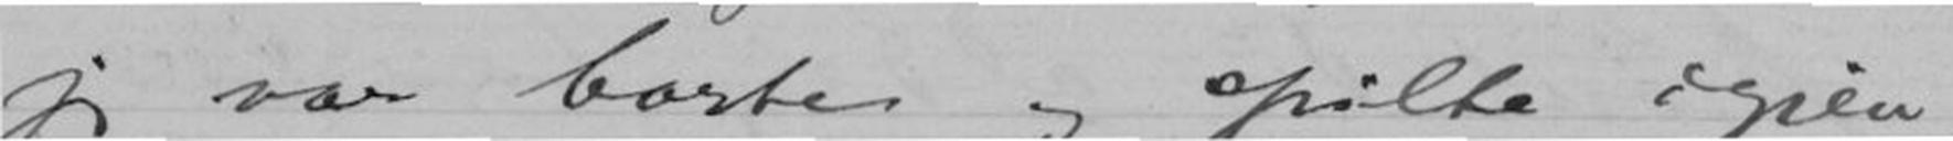jeg var borte og spilte igjen.
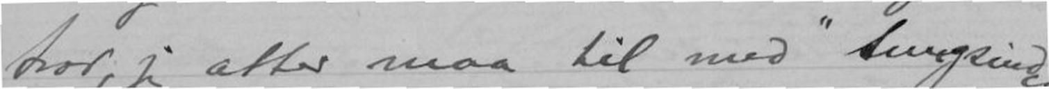tror, jeg atter maa til med "tungsindig
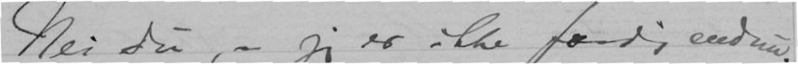Nei Du, - jeg er ikke færdig endnu,
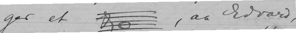ger et, aa Edvard,
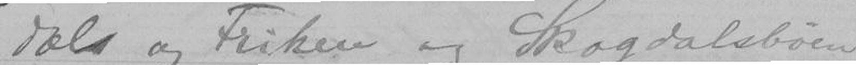dal og Friken og Skogdalsbøen
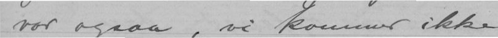vor ogsaa, vi kommer ikke
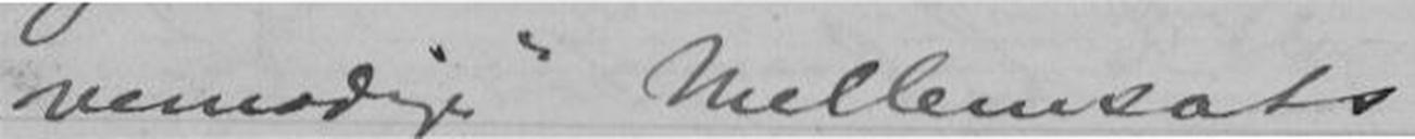vemodige" Mellemsats
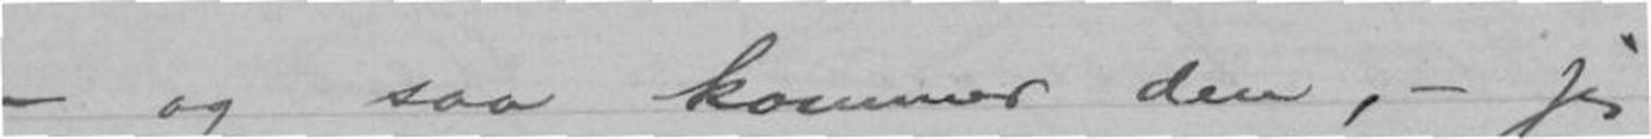- og saa kommer den, - jeg
